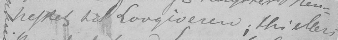nesket til Lovgiveren; thi ellers
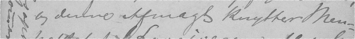og denne Afmagt knytter Men-
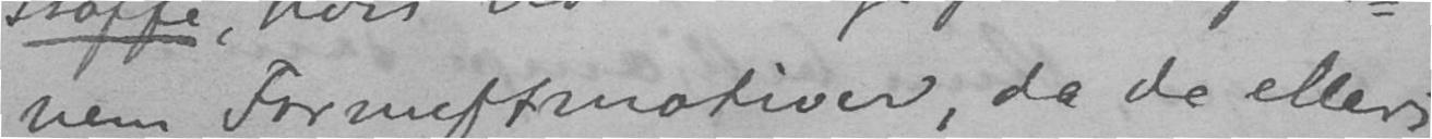nem Fornuftmotiver, da de ellers
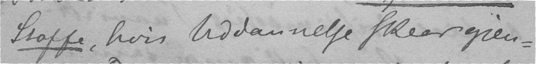Stoffe, hvis Uddannelse skeer gjen-
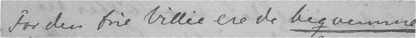For den frie Villie ere de beqvemme
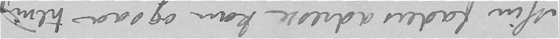Min faders adresse kan ogsaa til mig
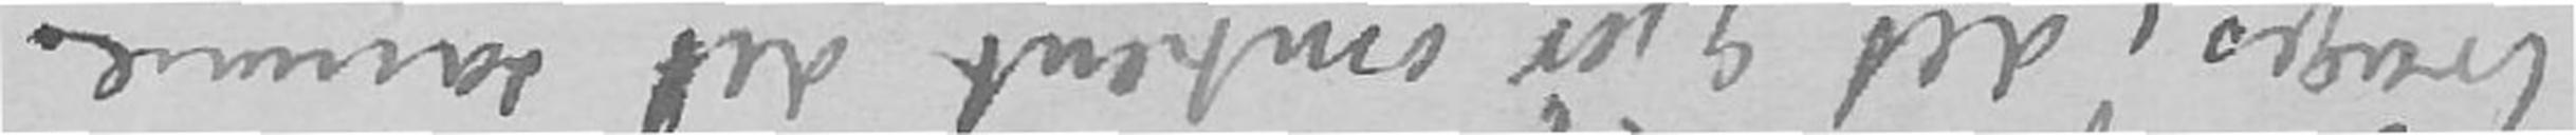bruges, det gjør omtrent det samme.
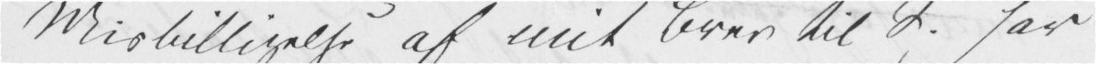Misbilligelse af mit Brev til S. har
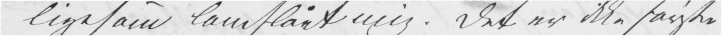ligesom lamslået mig. Det er ikke første
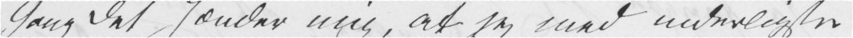Gang det hænder mig, at jeg med inderligste
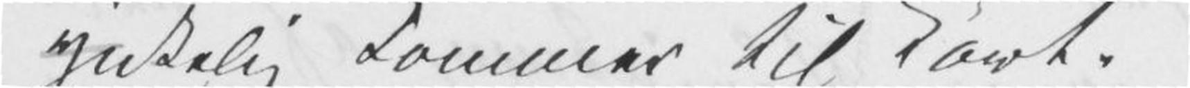ynkelig kommer til Kort.
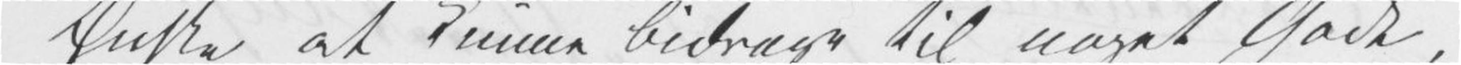Ønske at kunne bidrage til noget Gode,
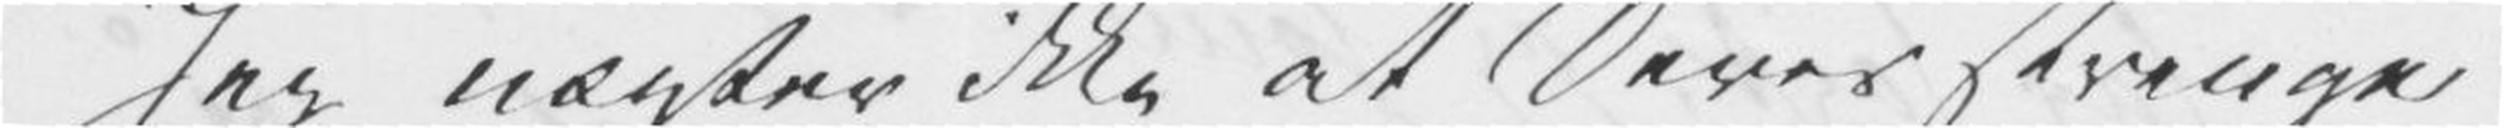Jeg nægter ikke at Deres strenge
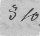§ 10.
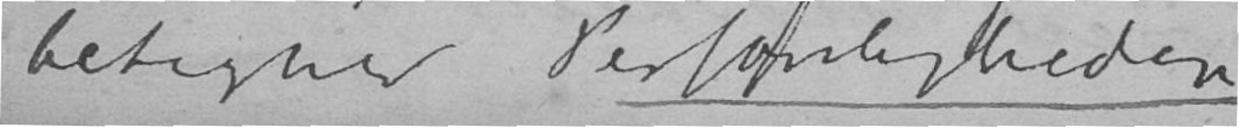betegner Personligheden
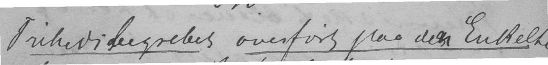Frihedsbegrebet overført paa den Enkelte
